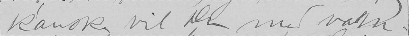kanske vil Du med væm-
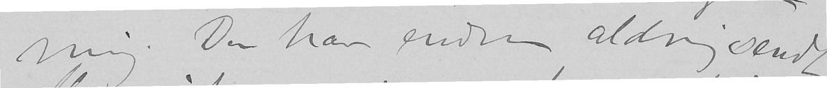mig. Du har endnu aldrig sendt
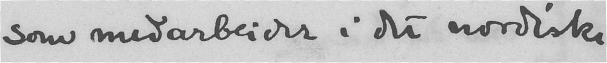som medarbeider i det nordiske
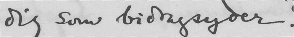dig som bidragsyder?
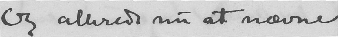Og allerede nu at nævne
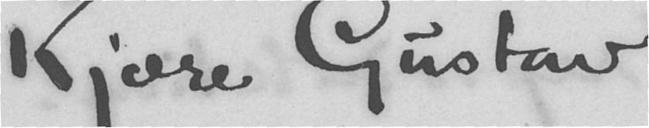Kjære Gustav
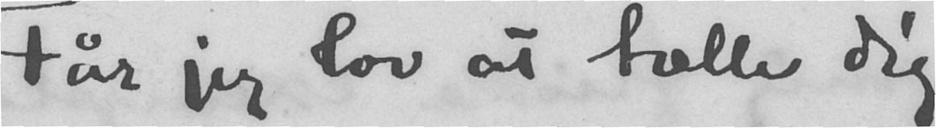Får jeg lov at tælle dig
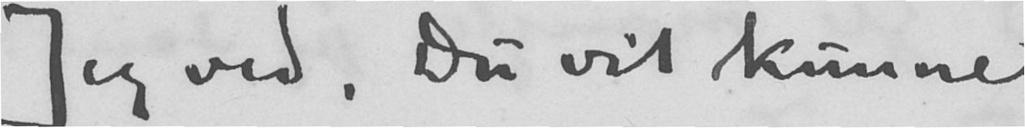Jeg ved, Du vil kunne
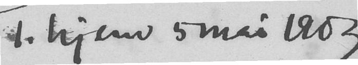T.hjem 5 mai 1903.
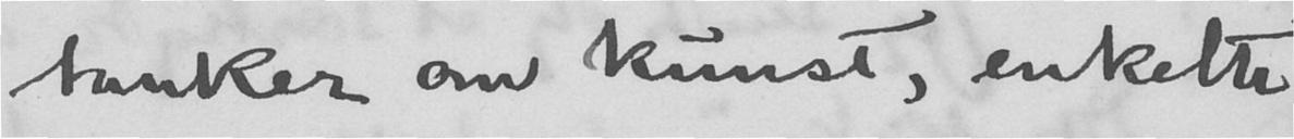tanker om kunst, enkelte
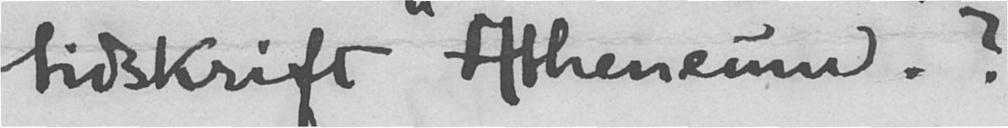tidsskrift "Atheneum"?
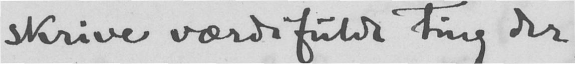skrive værdifulde ting der,
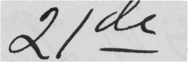21de
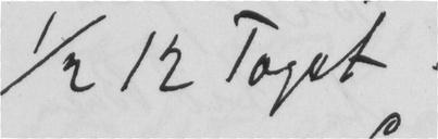1/2 12 Toget.
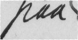paa
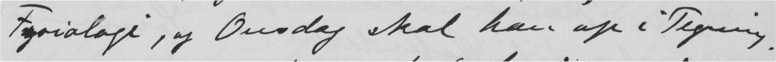Fysiologi, og Onsdag skal han op i Tegning.
